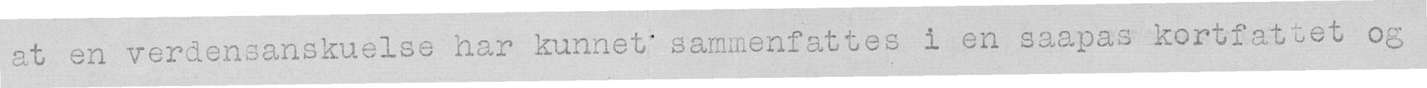at en verdensanskuelse har kunnet sammenfattes i en saapas kortfattet og
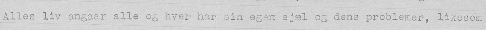Alles liv angaar alle og hver har sin egen sjæl og dens problemer, likesom
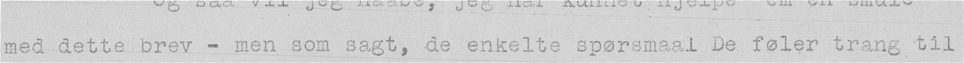med dette brev - men som sagt, de enkelte spørsmaal De føler trang til
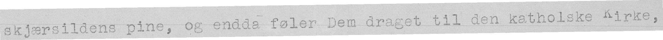skjærsildens pine, og endda føler Dem draget til den katholske Kirke,
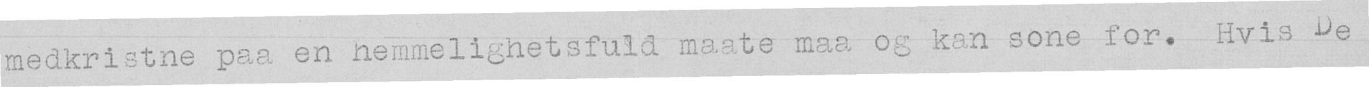medkristne paa en hemmelighetsfuld maate maa og kan sone for. Hvis De
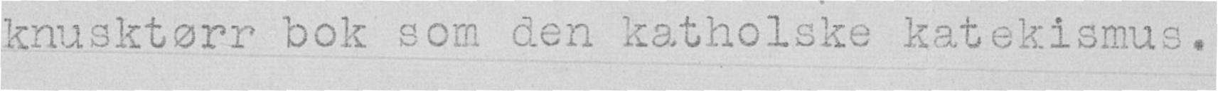knusktørr bok som den katholske katekismus.
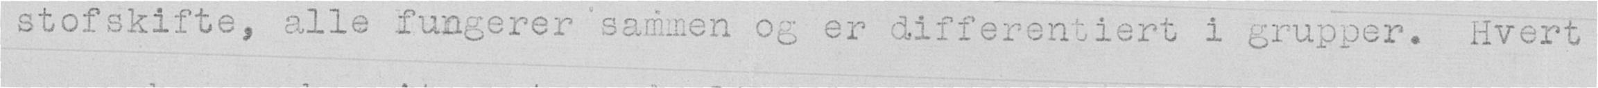stofskifte, alle fungerer sammen og er differentiert i grupper. Hvert
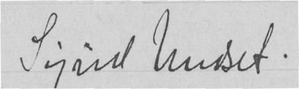Sigrid Undset.
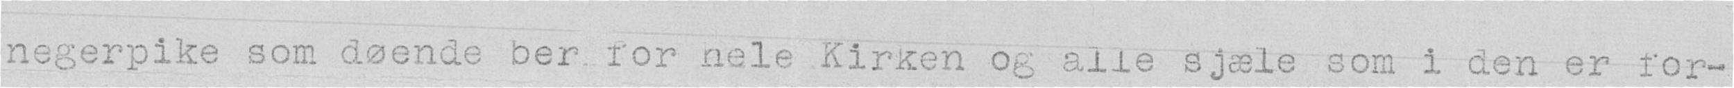negerpike som døende ber for hele Kirken og alle sjæle som i den er for¬
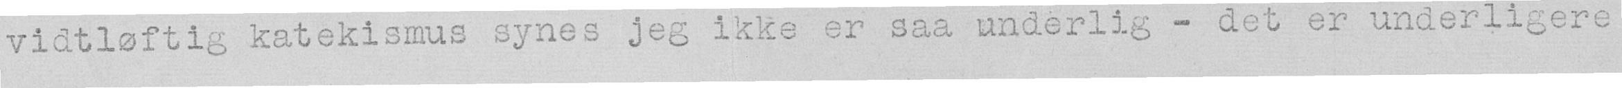vidtløftig katekismus synes jeg ikke er saa underlig - det er underligere
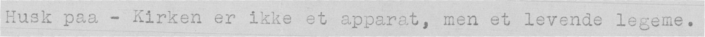Husk paa - Kirken er ikke et apparat, men et levende legeme.
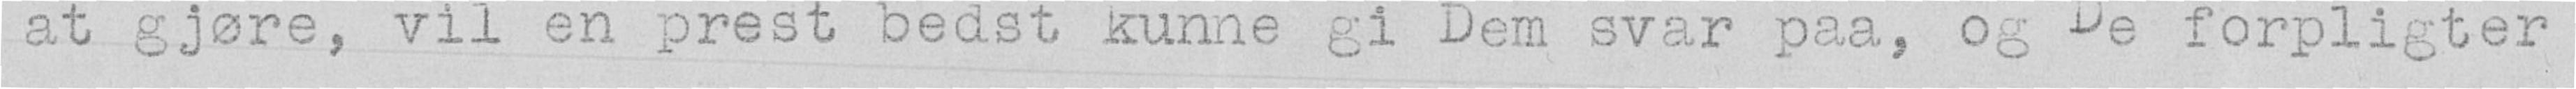at gjøre, vil en prest bedst kunne gi Dem svar paa, og De forpligter
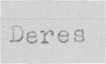Deres
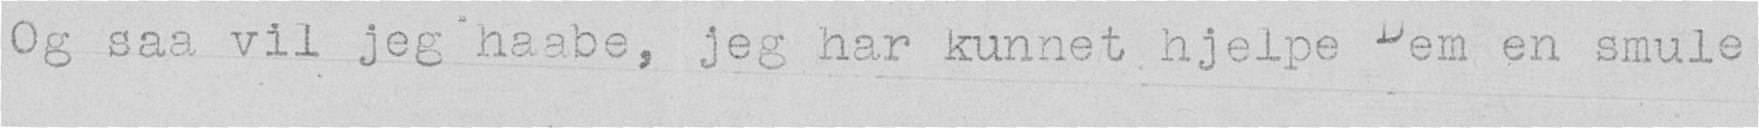Og saa vil jeg haabe, jeg har kunnet hjelpe Dem en smule
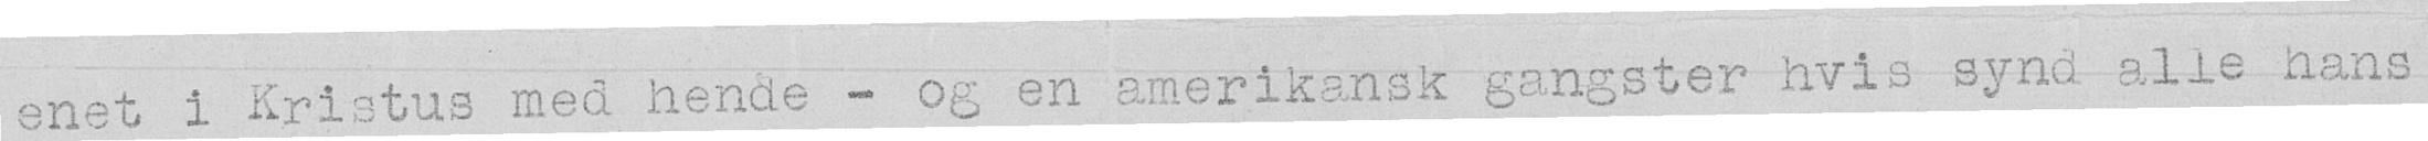enet i Kristus med hende - og en amerikansk gangster hvis synd alle hans
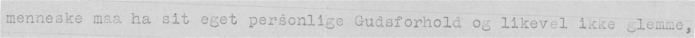menneske maa ha sit eget personlige Gudsforhold og likevel ikke glemme,
